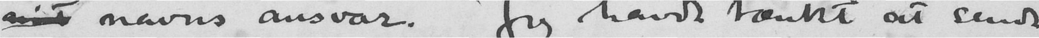mit navns ansvar. Jeg havde tænkt at sende
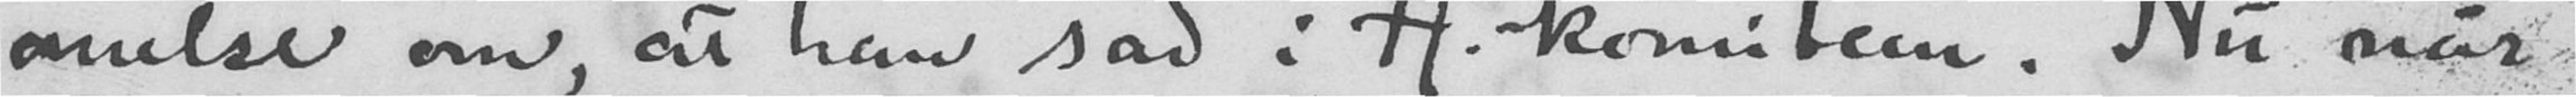anelse om, at han sad i A.komiteen. Nu når
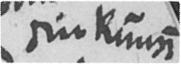din kunst
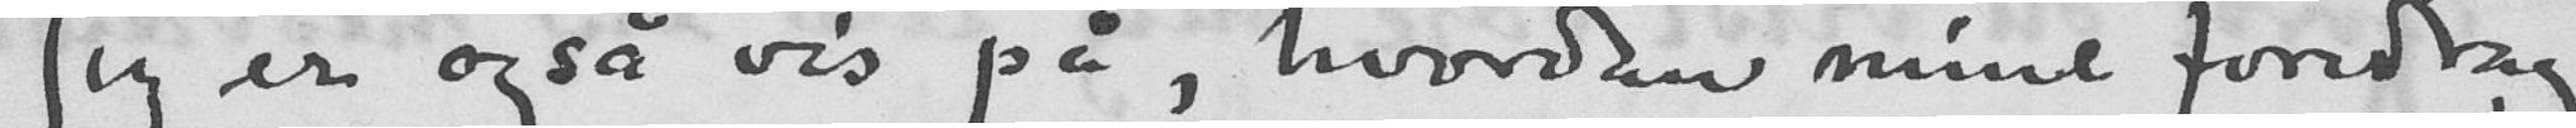Jeg er også vis på, hvordan mine foredrag
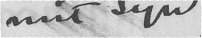mit syn
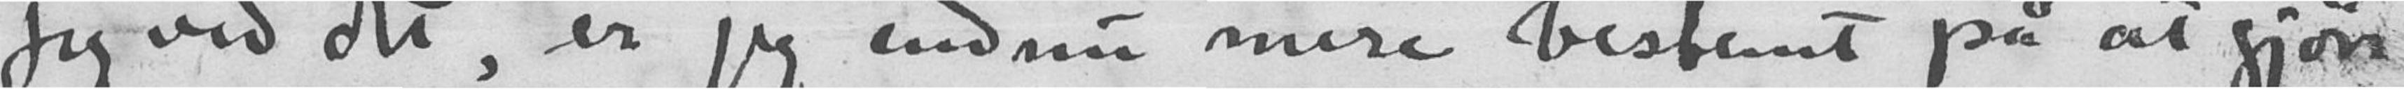jeg ved det, er jeg endnu mere bestemt på at gjøre
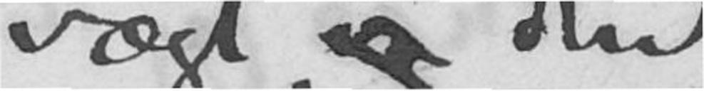vægt, og den
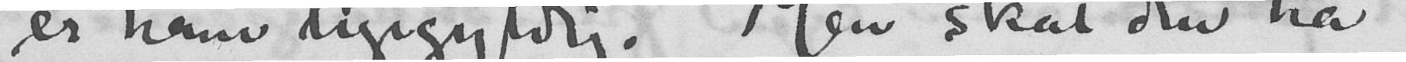er ham ligegyldig. Men skal den ha
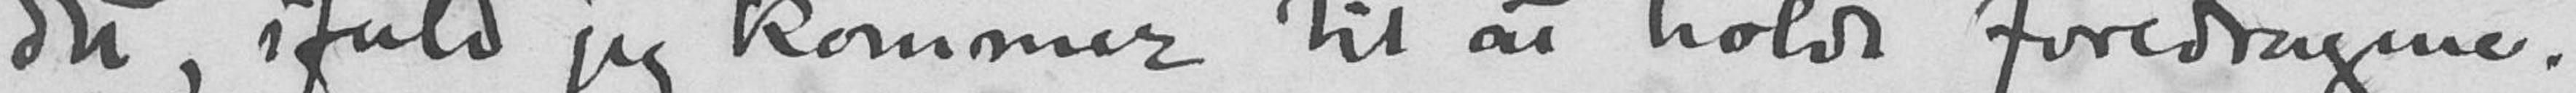det, ifald jeg kommer til at holde foredragene.
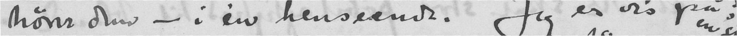hører dem - i en henseende. Jeg er vis på,
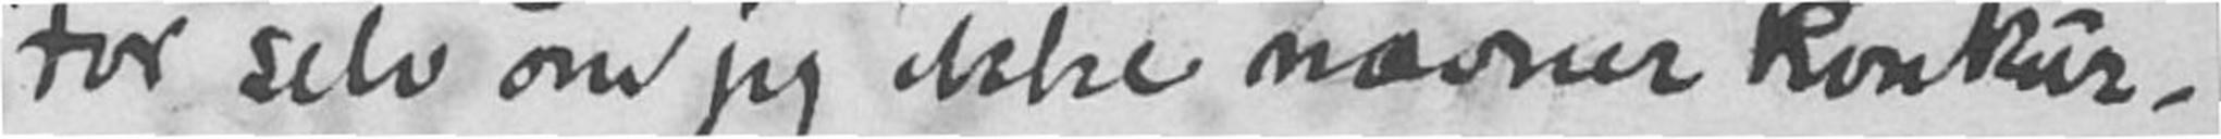For selv om jeg ikke nævner konkur-
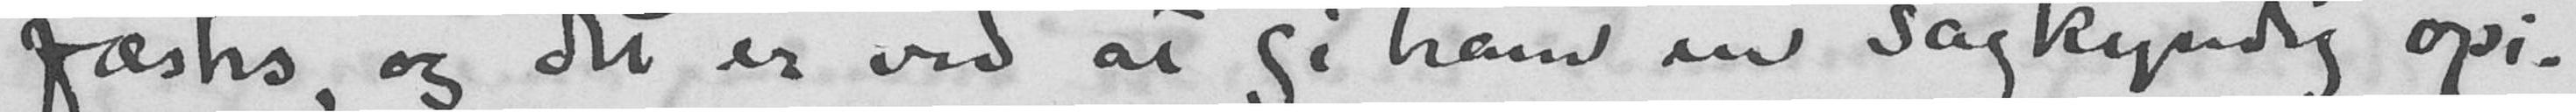fæstes, og det er ved at gi ham en sagkyndig opi-
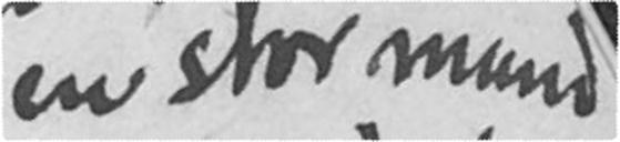en stor mand
